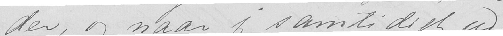der, og naar jeg samtidigt ud-
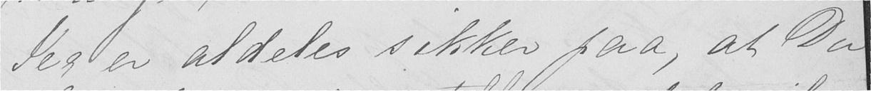Jeg er aldeles sikker paa, at Du
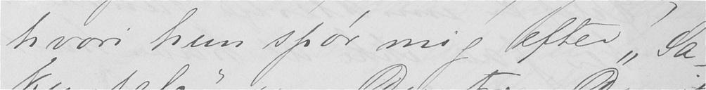hvori hun spør mig efter "Sa-
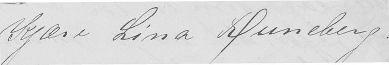Kjære Lina Runeberg.
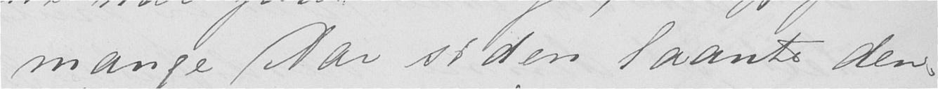mange Aar siden laante den.
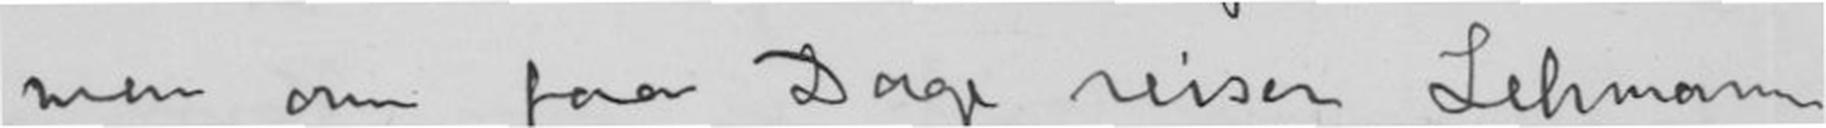men om faa Dage viser Lehmann,
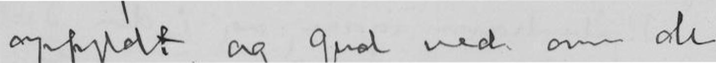opfyldt, og Gud ved om de
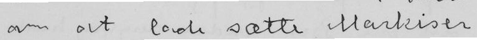om at lade sætte Markiser
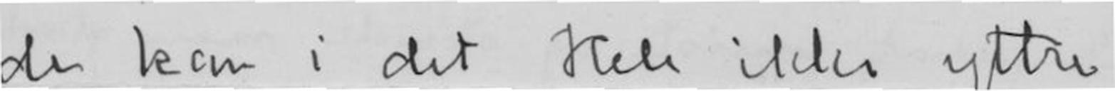de kan i det hele ikke yttre
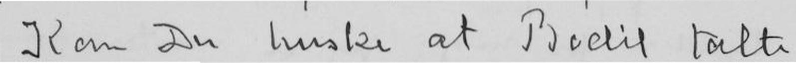Kan Du huske at Bodil talte
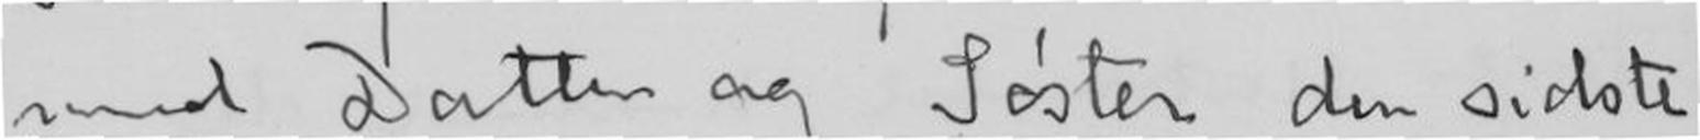med Datter og Søster den sidste
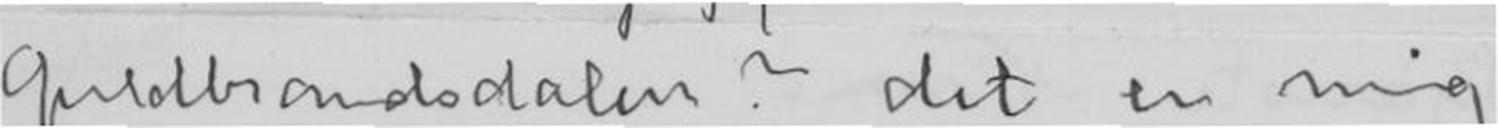Guldbrandsdalen? det er mig
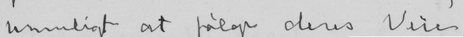hemmligt at følge deres Veier.
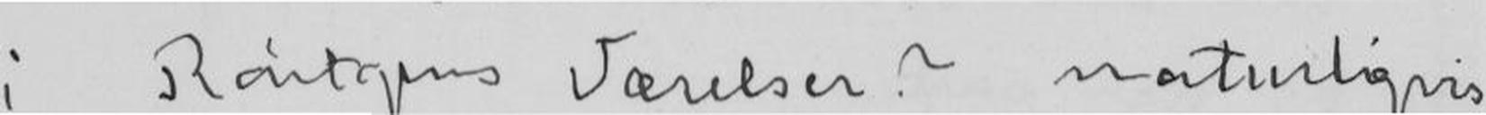i Röntgens Værelse? naturligvis
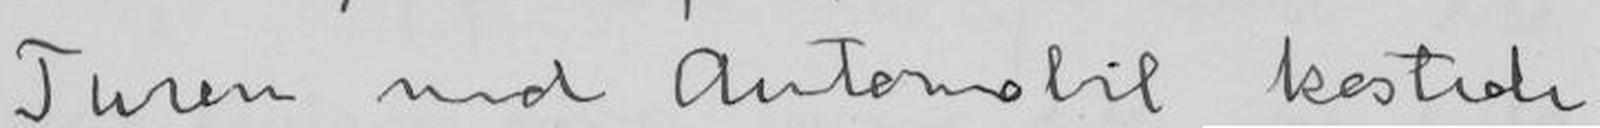Turen med Automobli kostede
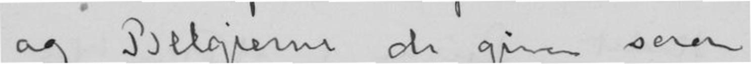og Belgierne, de give saa
